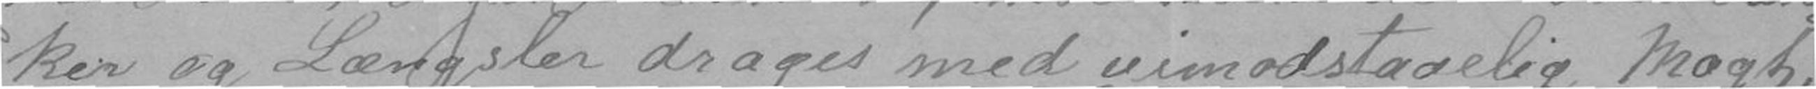ker og Længsler drages med uimodstaaelig Magt,
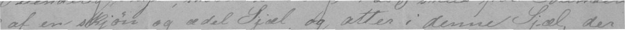af en skjøn og ædel Sjæl og atter i denne Sjæl der
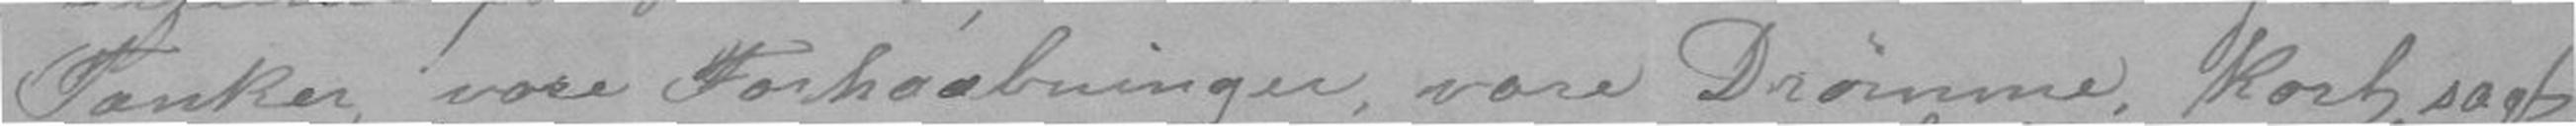Tanker, vore Forhaabninger, vore Drømme, kort sagt
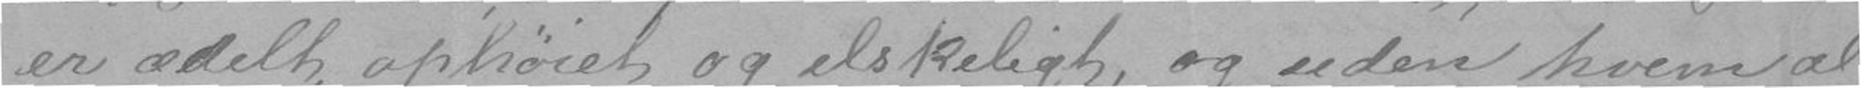er ædelt, ophøiet og elsket, og uden hvem al
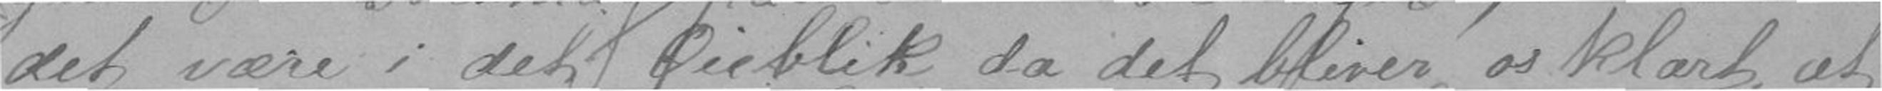det være i det Øieblik da det bliver os klart at
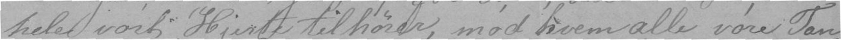hele vort Hjerte tilhører, mod hvem alle vore Tan-
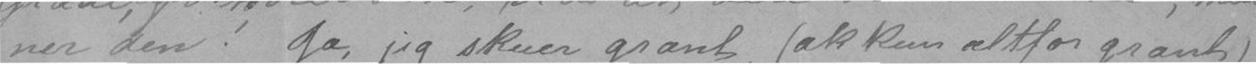ner den! Ja, jeg skuer grant, (ak kun altfor grant]
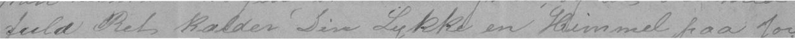fuld Ret kalder Din Lykke en Himmel paa Jor-
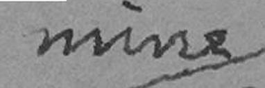mine
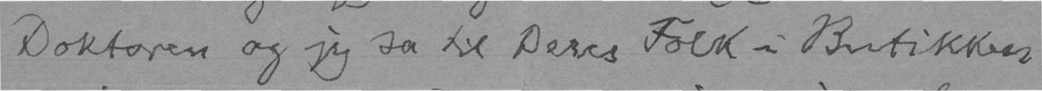Doktoren og jeg sa til Deres Folk i Butikken
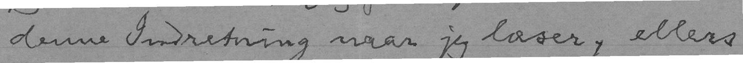denne Indretning naar jeg læser, ellers
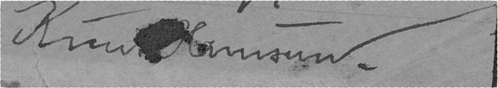Knu  amsun.
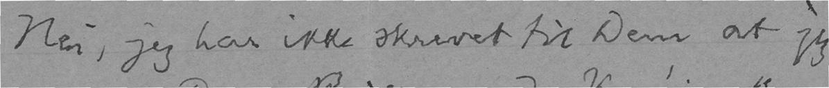Nei, jeg har ikke skrevet til Dem at jeg
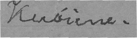Kuøiene.
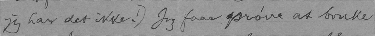jeg har det ikke!) Jeg faar prøve at bruke
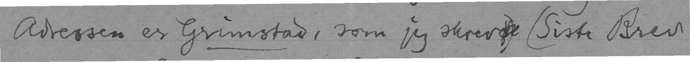Adressen er Grimstad, som jeg skreve)e (Siste Brev
